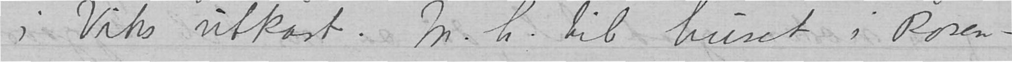i Viks utkast.  M.h. til huset i Rosen-
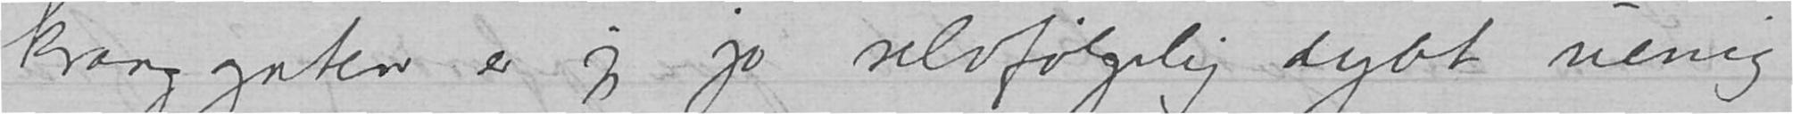kranzgaten er jeg jo selvfølgelig dybt uenig
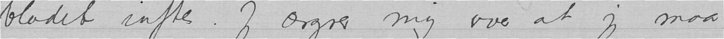bladet iaftes. Jeg ærgrer mig over at jeg maa
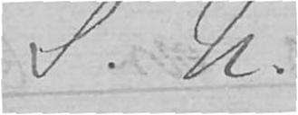S.U.
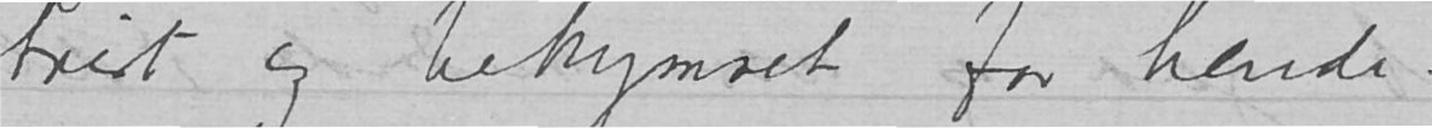trist og bekymret for hende.
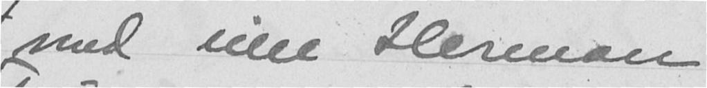med lille Herman
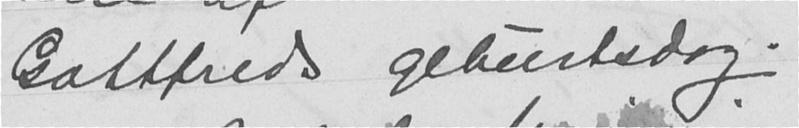Gottfreds geburtsdag
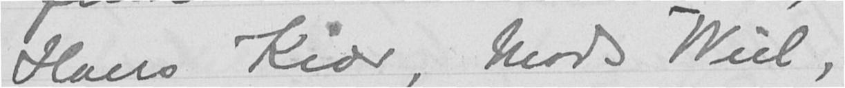Hans Kiær, Mads Wiel,
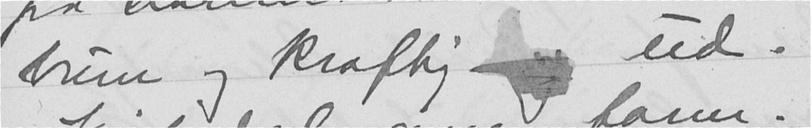brun og kraftig og ud.
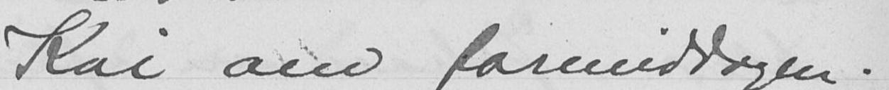Kai om formiddagen
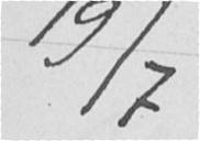19/7
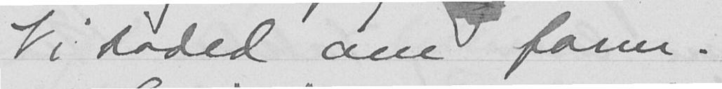Vi baded om form.
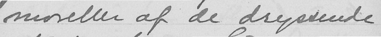moreller af de dryssende
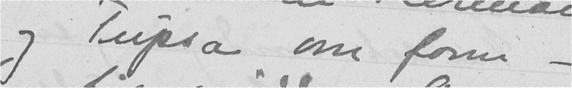og Tupsa om form -
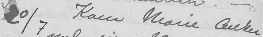20/7 Kom Marie Anker
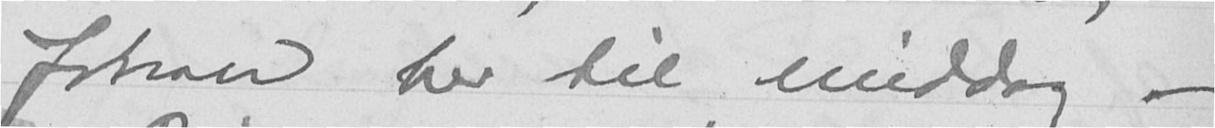Johan her til middag -
